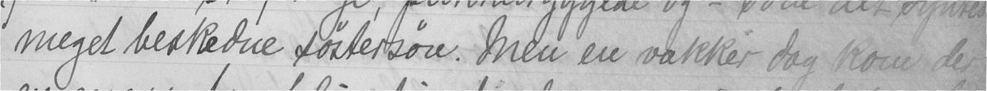meget beskedne søstersøn. Men en vakker dag kom der
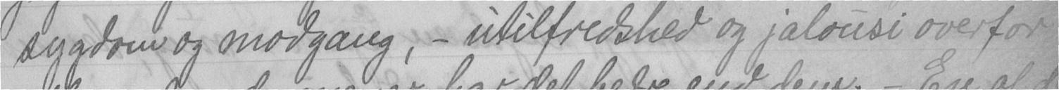sygdom og modgang, - utilfredshed og jalousi overfor
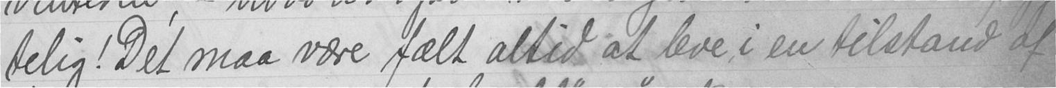telig. Det maa være fælt altid at leve i en tilstand af
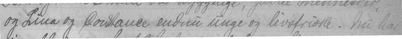og Lina og Constance endnu unge og livsfriske. Nu har
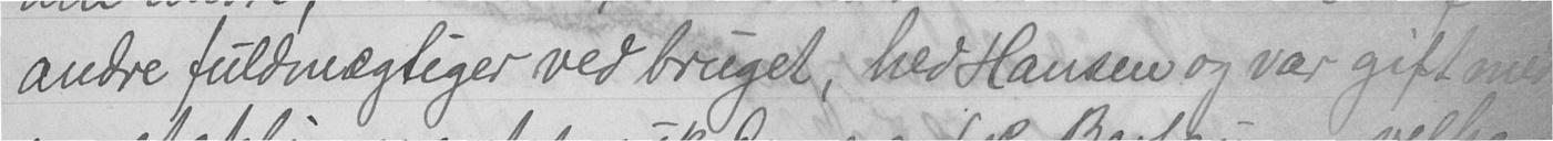andre fuldmægtiger ved bruget, hed Hansen og var gift med
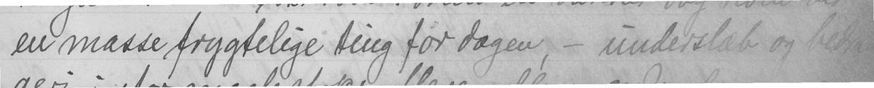en masse frygtelige ting for dagen, - underslæb og bedra-
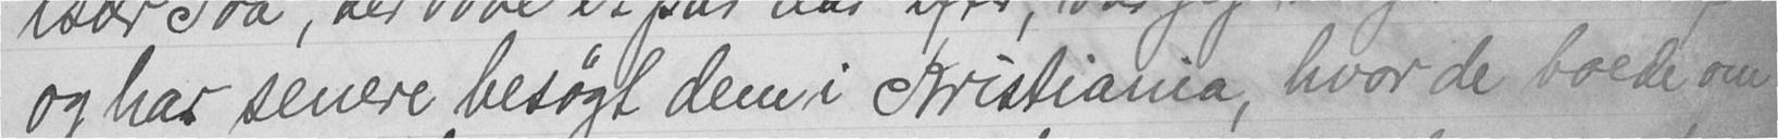og har senere besøgt dem i Kristiana, hvor de boede om
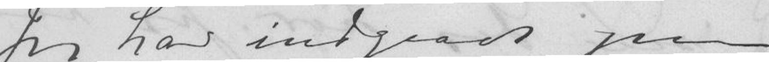Jeg har indgaadt paa
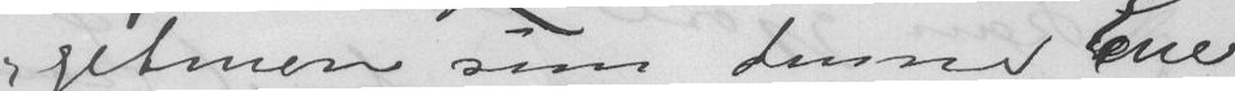getmere som denne Ene
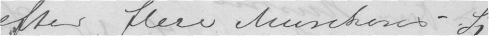efter flere Musikeres Si-
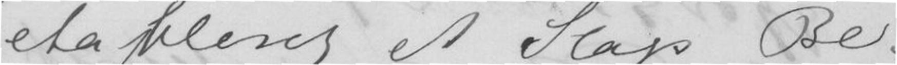etableret et Slags Be-
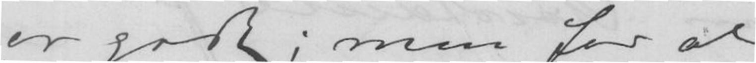er godt; men for at
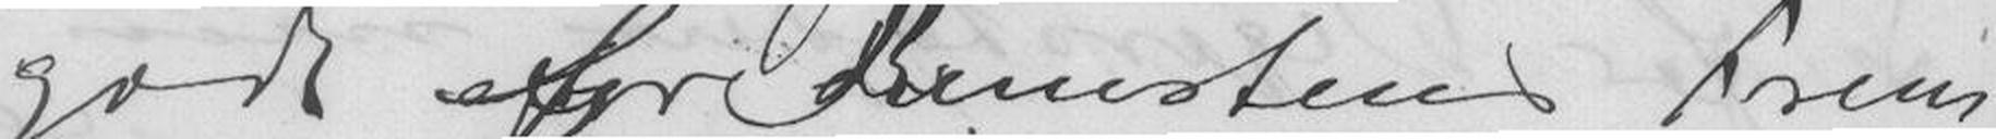godt for Kunstens Frem-
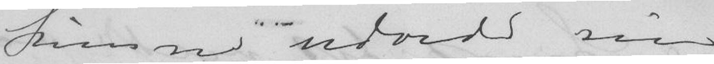kunne udvide sin
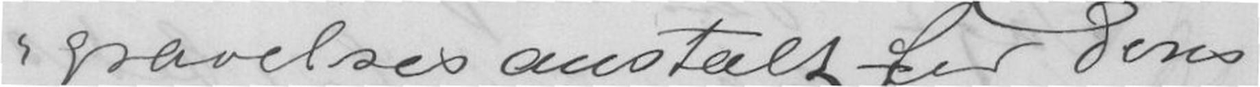gravelses anstalt for Deres
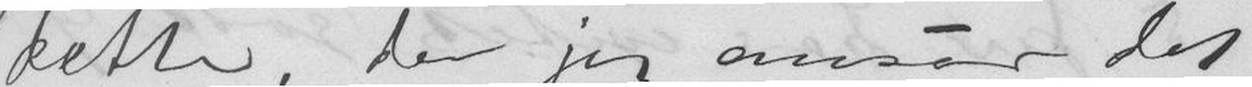dette, der jeg anser det
